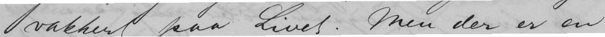vakkert paa Livet. Men der er en
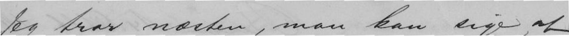Jeg tror næsten, man kan sige at
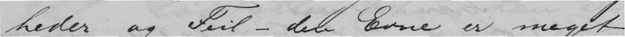heder og Feil - den Evne er meget
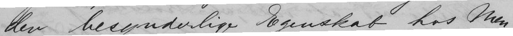den besynderlige Egenskab hos Men-
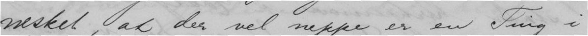nesket, at der vel neppe er en Ting i
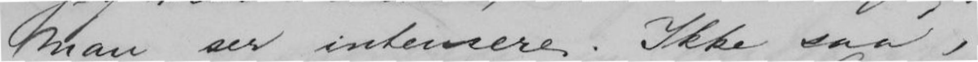Man ser intensere. Ikke saa,
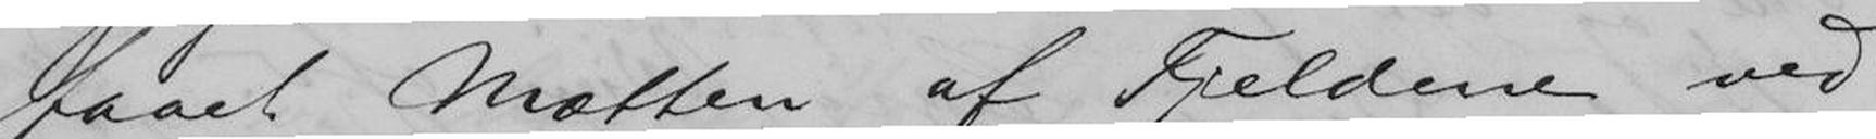faaet Mætten af Fjeldene ved
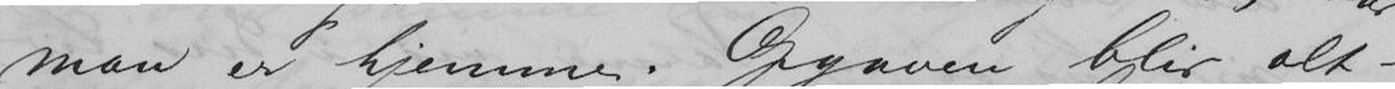man er hjemme. Opgaven blir alt-
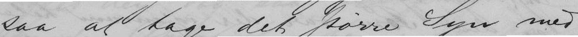saa at tage det større Syn med
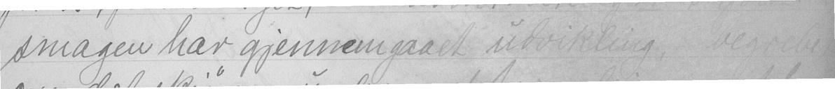smagen har gjennemgaaet udvikling, begrebet
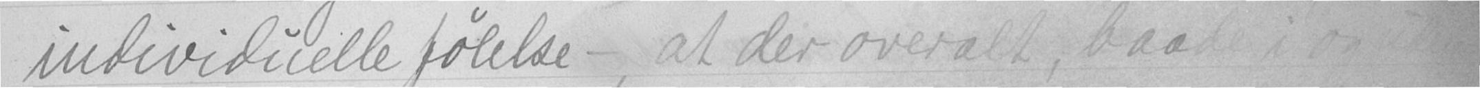individuelle følelse -, at der overalt, baade i og uden-
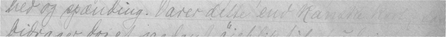hed og spænding. Varer dette end kanske kort, saa
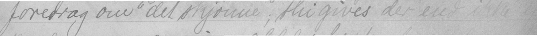foredrag om "det skjønne"; thi gives, der end ikke
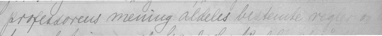professorens mening aldeles bestemte regler og
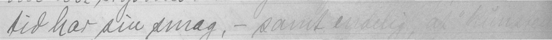tid har sin smag, - samt endelig at kunsten
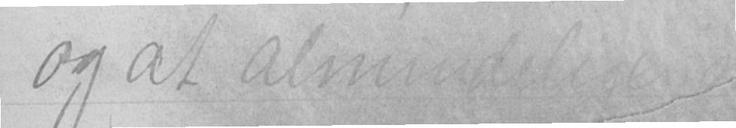og at almindelig
